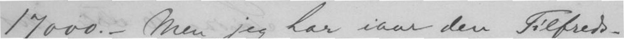17000.- Men jeg har iaar den Tifreds-
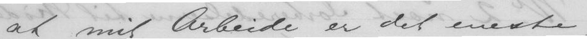at mit Arbeide er det eneste,
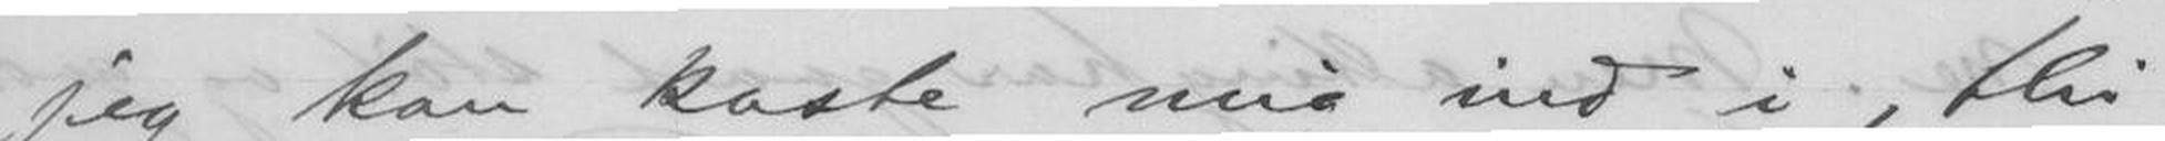jeg kan kaste mig ind i, thi
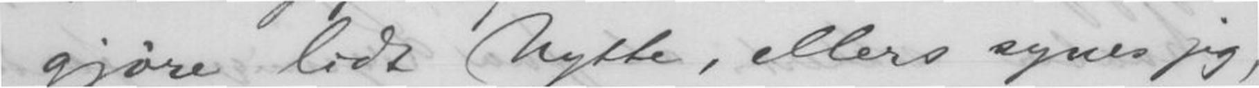gjøre lidt Nytte, ellers synes jeg,
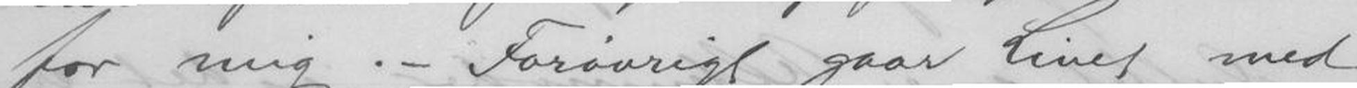for mig. - Forøvrigt gaar Livet med
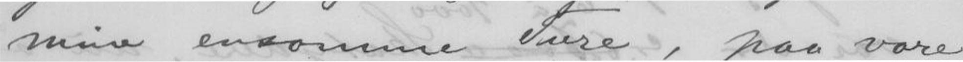mine ensomme Ture, paa vore
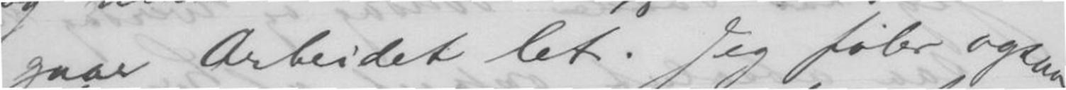gaar Arbeidet let. Jeg føler ogsaa
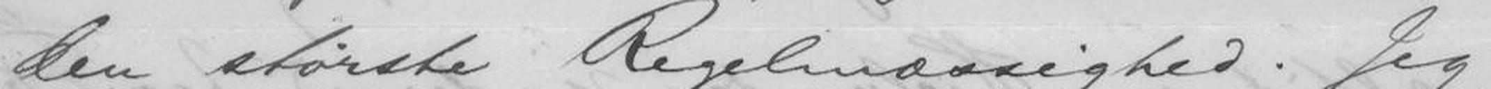den største Regelmæssighed. Jeg
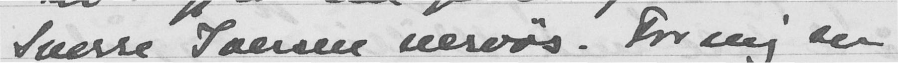Sverre Hansen nervøs. For mig ser
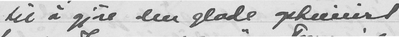til å gjøre den glade optimist
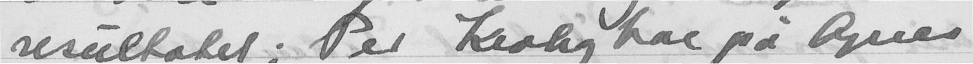resultatet; Per Krohg har på Agnes
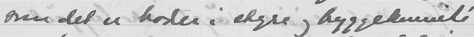som det er hoder i styre og byggekommitè
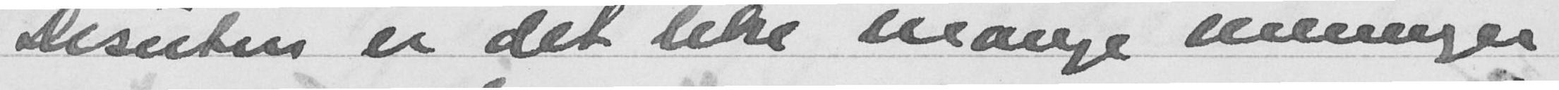Dessuten er det like mange meninger
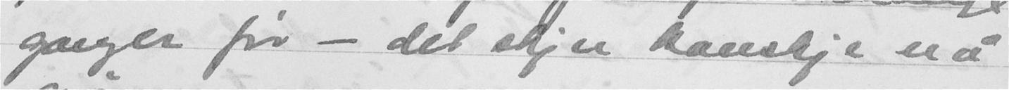ganger før - det skjer kanskje nå
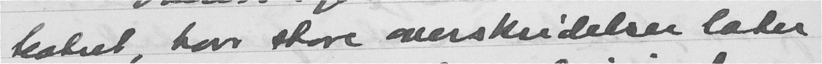teatret, hvor store overskridelser later
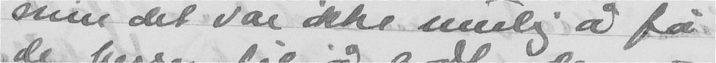men det var ikke mulig å få
